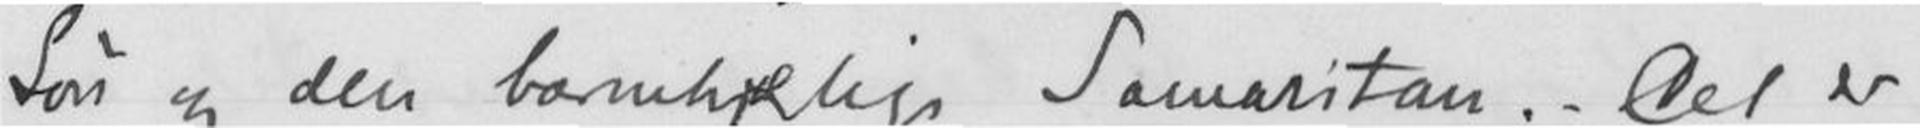Søn og den barmhjærtige Samaritan. Det er
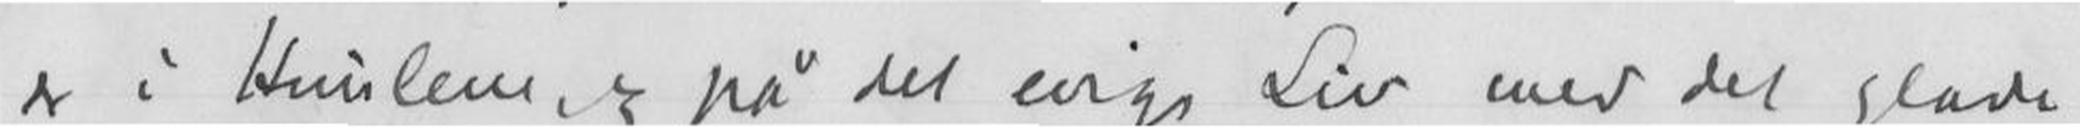er i Himlene, og på det evige Liv med det glade
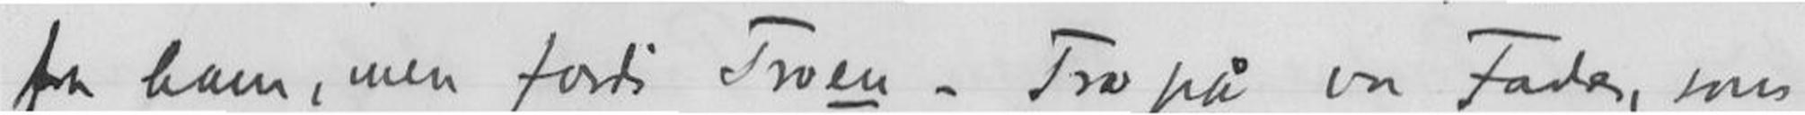fra ham, men fordi Troen - Tro på en Fader, som
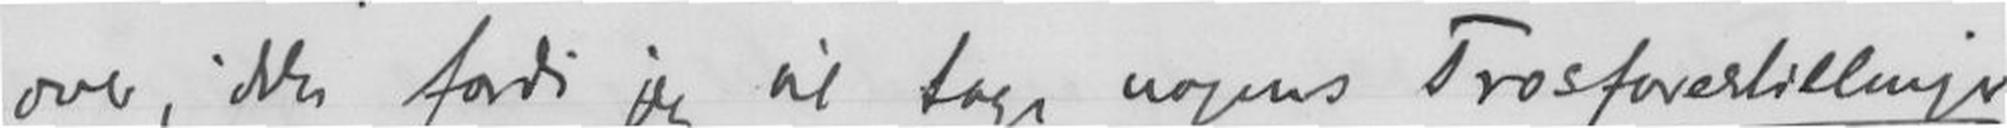over, ikke fordi jeg vil tage nogens Troforestillinger
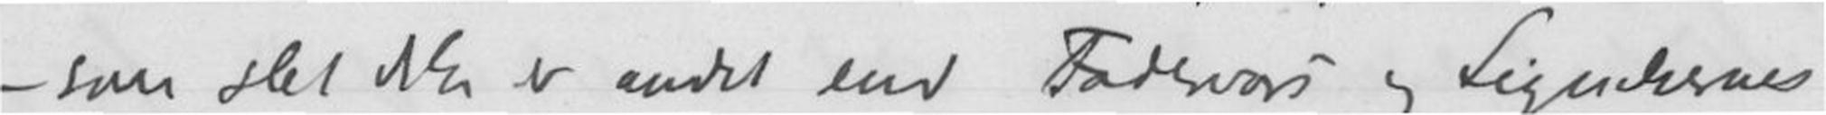- som slet ikke er andet end Fadervors og Lignelsernes
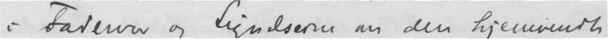i Fadevor og Lignelserne om den hjemvendt
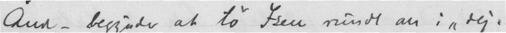Ånd - begynder at tø Isen rundt om i dej-
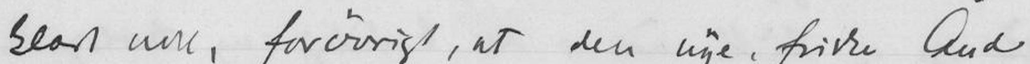klart nok, forøvrigt, at den nye, første Ånd
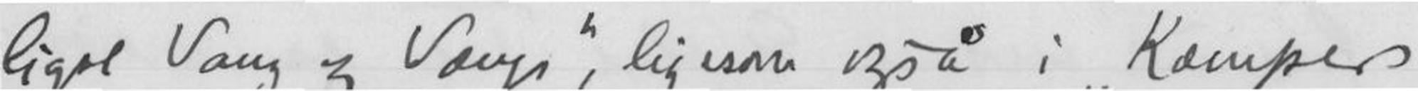ligt Sang og Sange, ligesom også i Kæmper
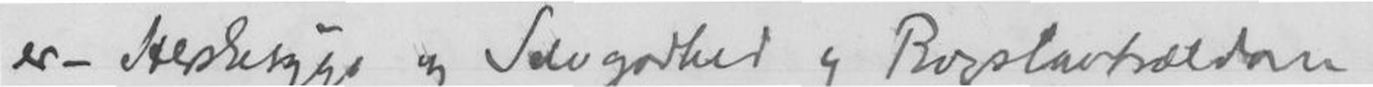er- Herskesyge og Selvgodhed og Bogstavtrældom
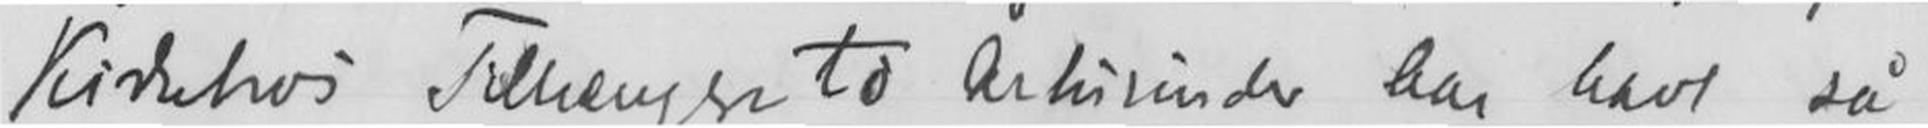Kirketros Tilhenger to Årtusinder har havt så
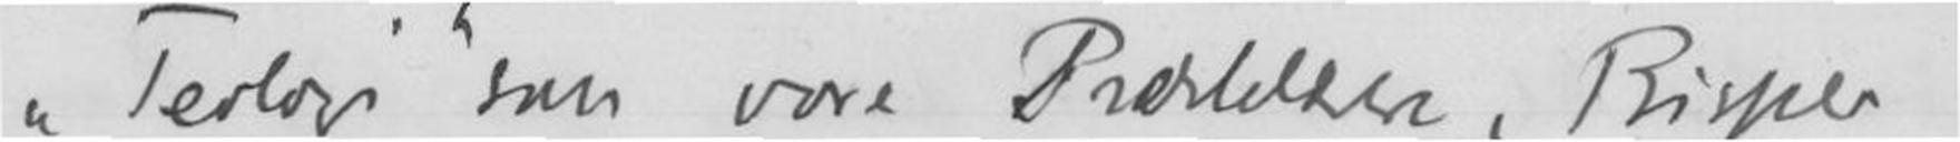Teologi som vore Prælater, Bisper
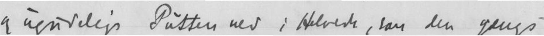og ugudelige Putten ned i Helvede, som den gangs
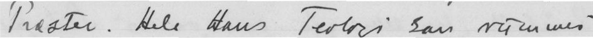Præster. Hele Hans Teologi kan rummes
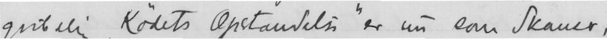gribelig Kødets Opstandelse er nu som Skauer,
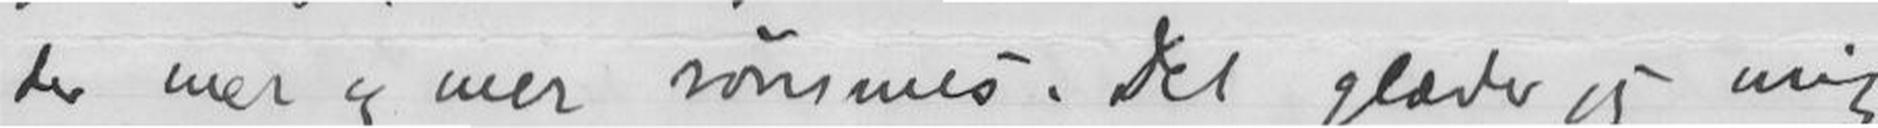der mer og mer rømmes. Det glæder jeg mig
